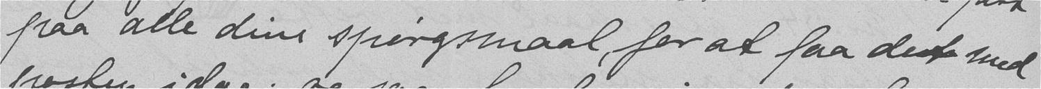paa alle dine spørgsmaal, for at faa det med
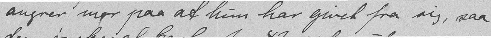angrer mor paa at hun har givet fra sig, saa
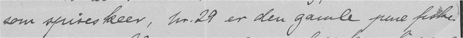som spiseskeer, nr. 29 er den gamle pene fiske-
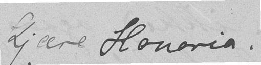Kjære Honoria!
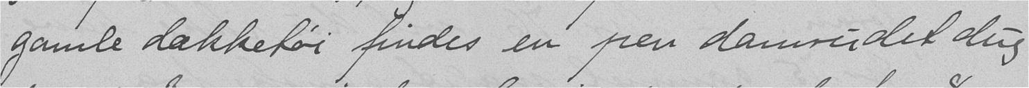gamle dækketøi findes en pen damrudet dug
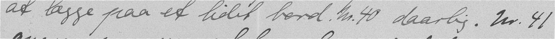at lægge paa et lidet bord. Nr.10 daarlig. Nr. 47
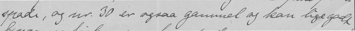spade, og nr. 30 er ogsaa gammel og kan lige godt
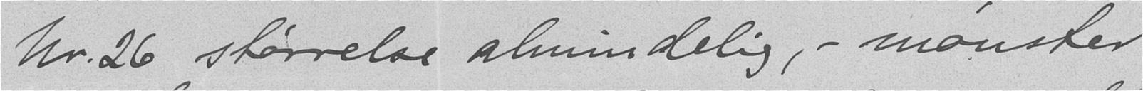Nr. 26 størrelse almindelig, - mønster
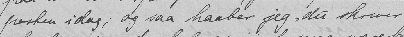posten i dag; og saa haaber jeg du skriver
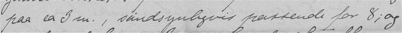paa ca 3 m., sandsynligvis passende for 8; og
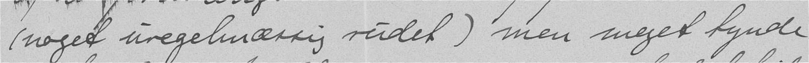(noget uregelmæssig rudet) men meget tynde
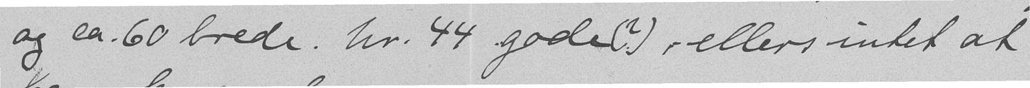og ca. 60 brede. Nr. 44 gode(?), ellers intet at
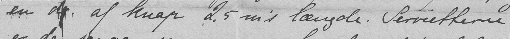en dg af knap 2.5 m's længde. Servietterne
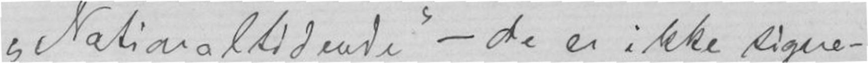Nationaltidende - de er ikke signe-
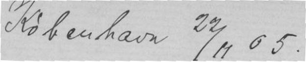København 22/11 05.
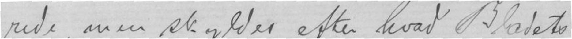rede, men skyldes efter hvad Bladets
